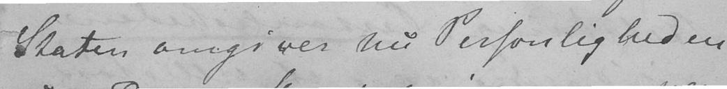Staten omgiver nu Personligheden
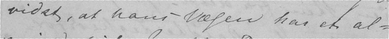vidst, at hans Væsen har et al-
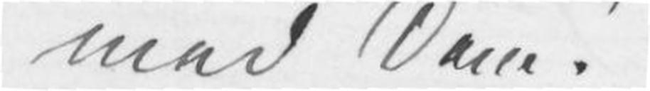med Dem!
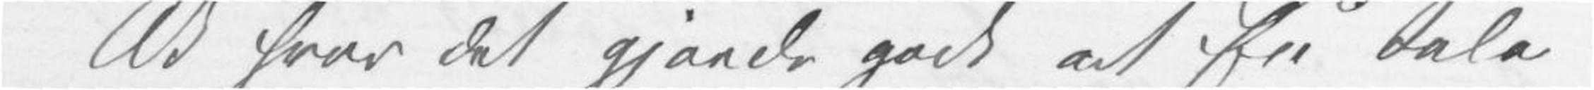Ak hvor det gjorde godt at få tale
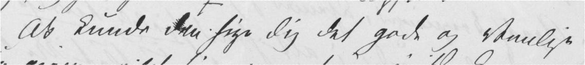Ak kunde den sige Dig det gode og Venlige
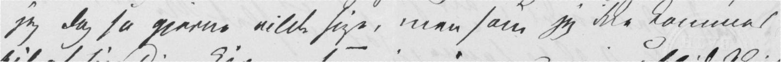jeg dog så gjerne vilde sige, men som jeg ikke kommer
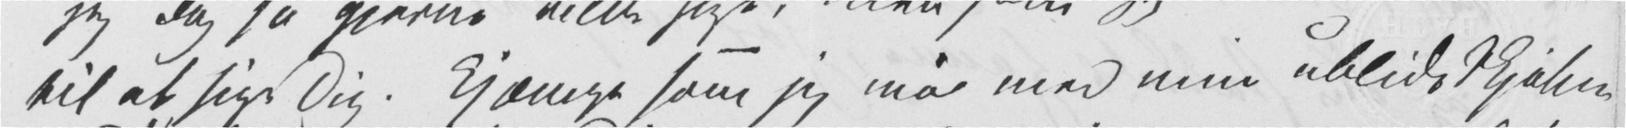til at sige Dig. Kjæmpe som jeg må med min ublide Skjæbne
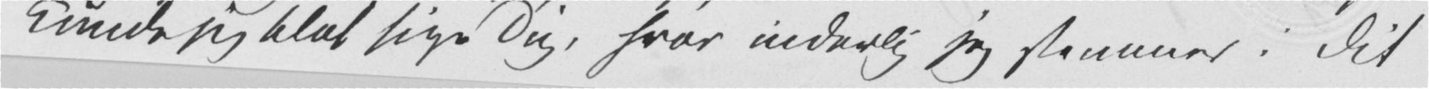kunde jeg blot sige Dig, hvor inderlig jeg stemmer i Dit
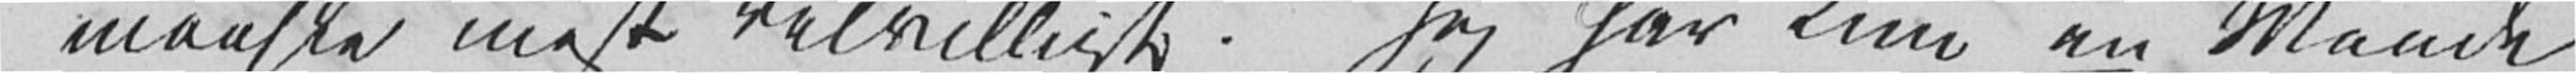maaske mest velvilligt. Jeg har kun en Maade
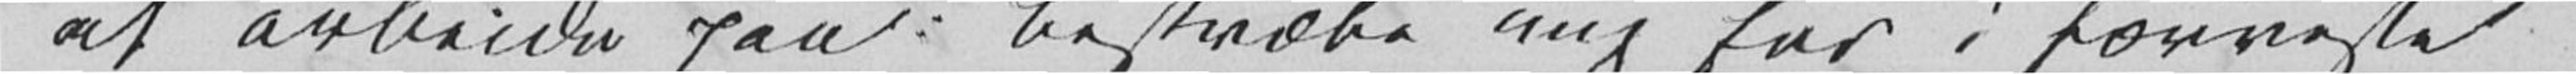at arbeide paa: bestræbe mig for i færreste
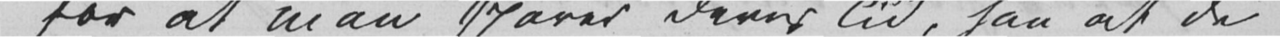for at man sparer deres Tid, saa at de
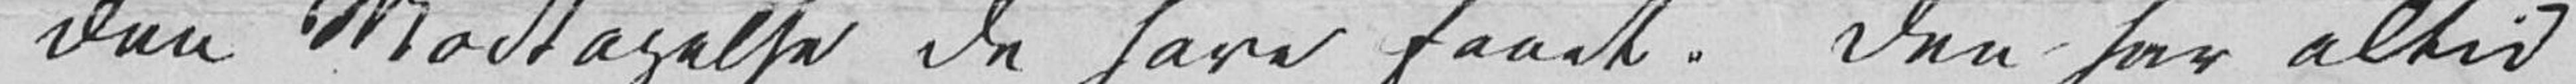den Modtagelse de have faaet. Den har altid
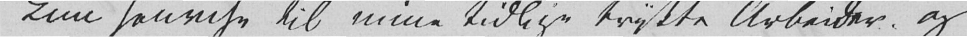kun henvise til mine tidligere trykte Arbeider, og
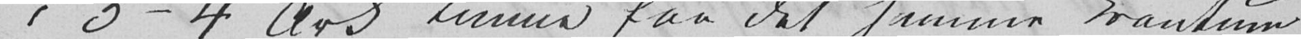i 3–4 Ark kunne faa det samme Kvantum
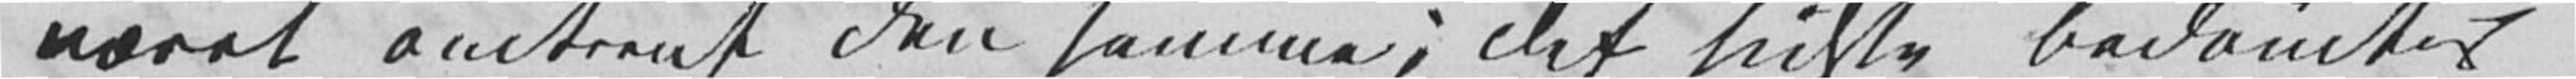været omtrent den samme; det sidste bedømtes
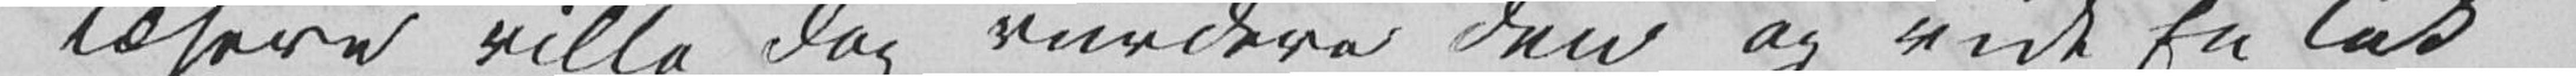Læsere ville dog vurdere den og vide En Tak
